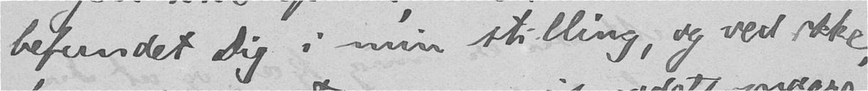befundet Dig i min stilling, og ved ikke
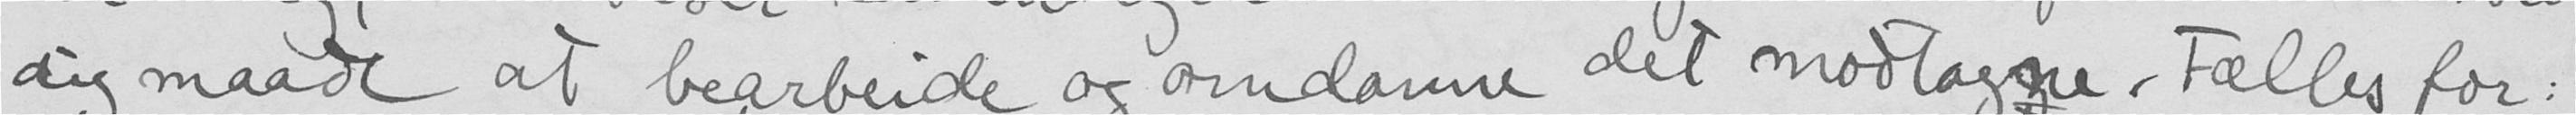dig maade at bearbeide og omdanne det modtagne. Fælles for
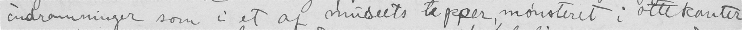indramninger som i et af museets tepper, mønsteret i ottekanter
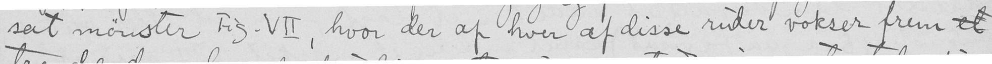seet mønster Fig. VII, hvor der af hver af disse ruder vokser frem
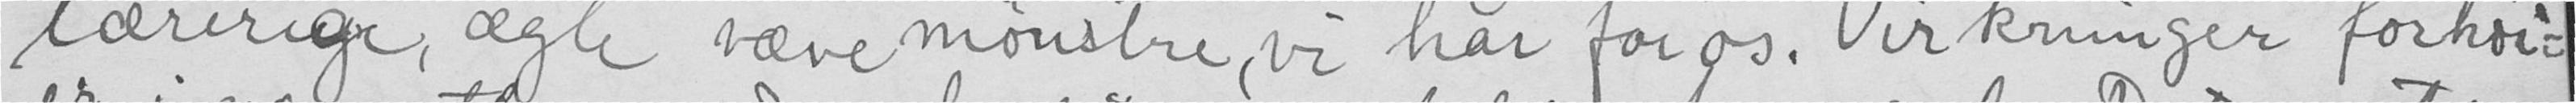lærerige, ægte vævemønstre, vi har for os. Virkninger forhøi-
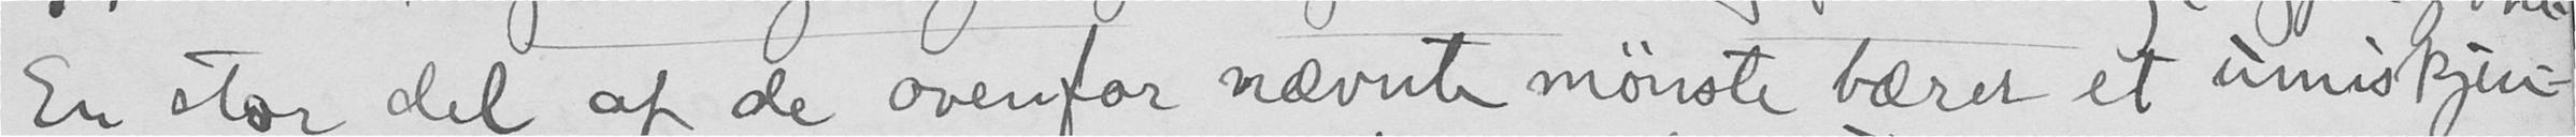En stor del af de ovenfor nævnte mønste bærer et umiskjen-
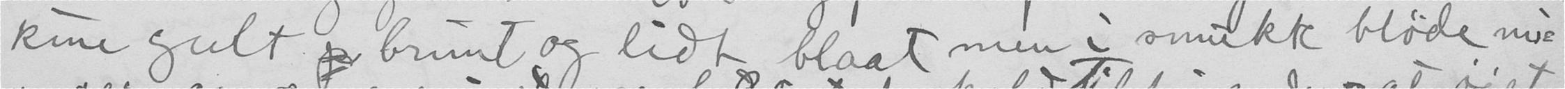kun gult, brunt og lidt blaat men i smukt bløde nu-
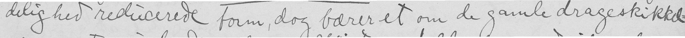delighed reducerede form, dog bærer et om de gamle drageskikkel-
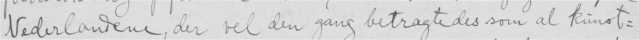Nederlandene, der vel den gang betragtedes som al kunst-
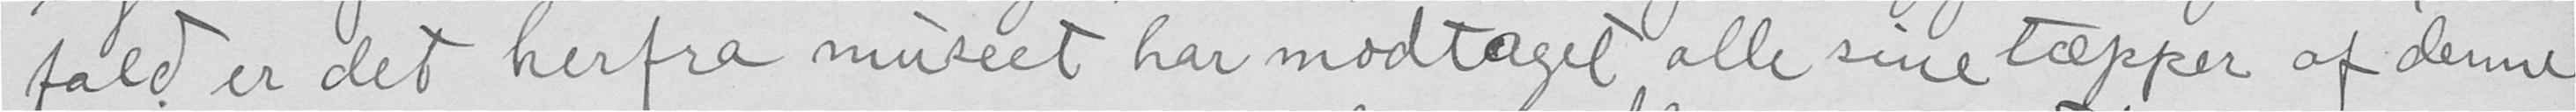fald er det herfra museet har modtaget alle sine tæpper af denne
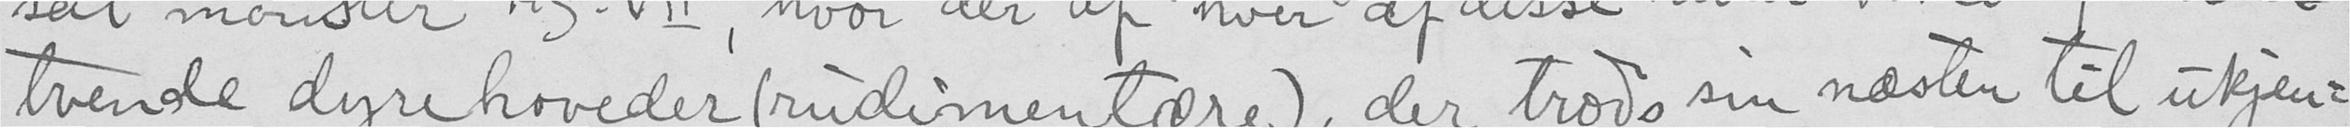tvende dyrehoveder (rudimentære), der trods sin næsten til ukjen-
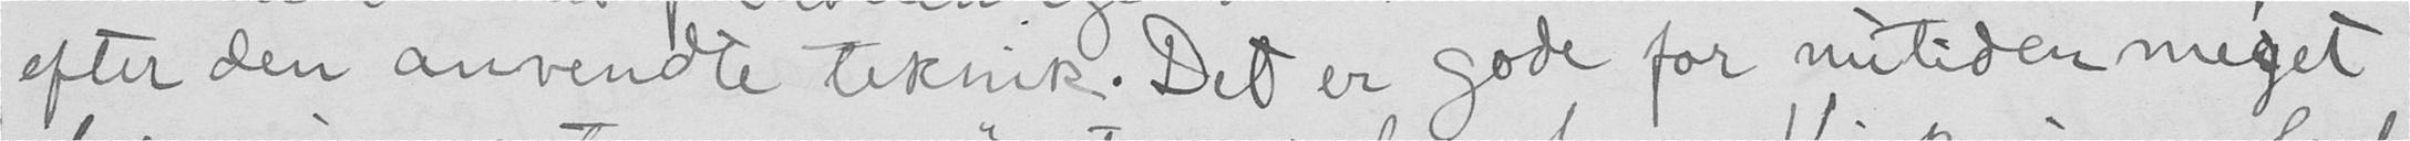efter den anvendte teknik. Det er gode for nutiden meget
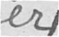er
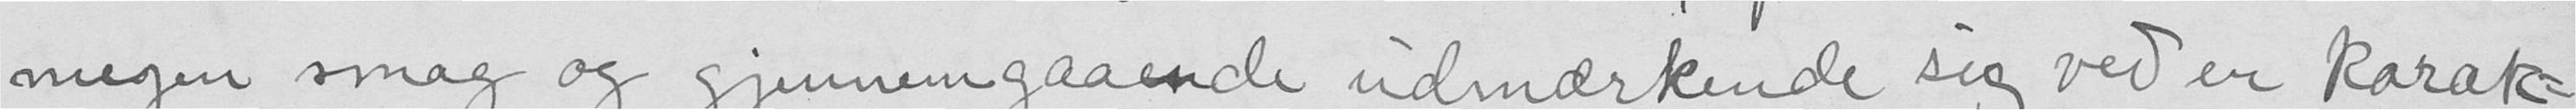megen smag og gjennemgaaende udmærkende sig ved en karak-
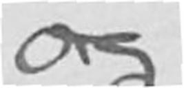og
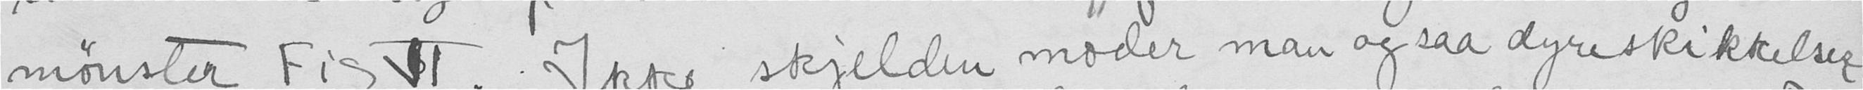mønster Fig VI. Ikke skjelden møder man og saa dyreskikkelsen
